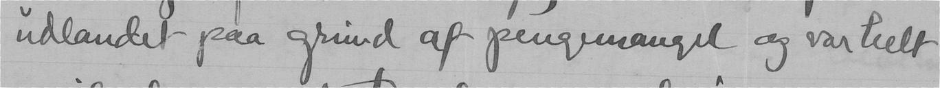udlandet paa grund af pengemangel og var helt
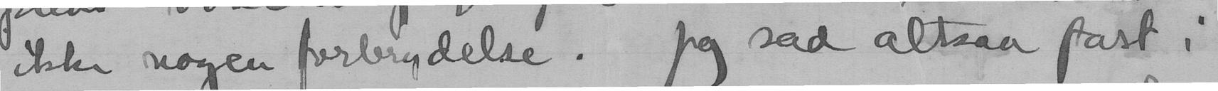ikke nogen forbrydelse. Jeg sad altsaa fast i
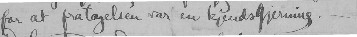for at fratagelsen var en kjendsgjerning. -
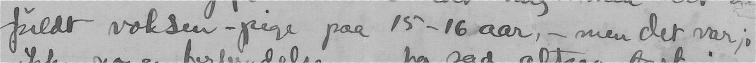fuldt voksen - pige paa 15-16 aar, - men det var jo
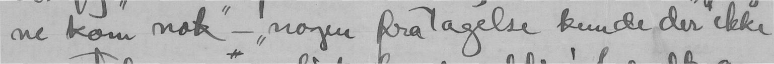ne kom nok" - "nogen fratagelse kunde der ikke
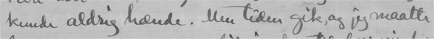kunde aldrig hænde. Men tiden gik, og jeg maatte
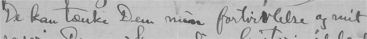De kan tænke Dem min fortvivlelse og mit
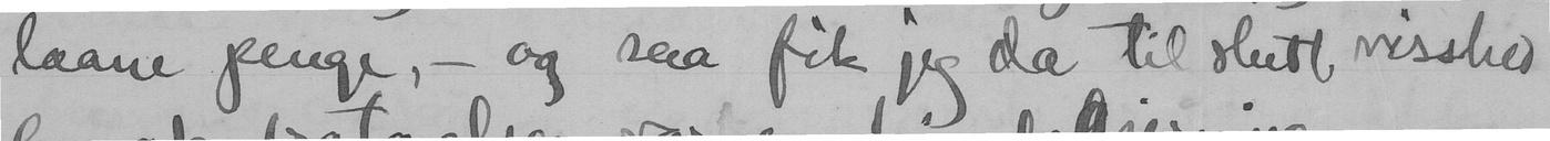laane penge, - og saa fik jeg da til slutt visshed
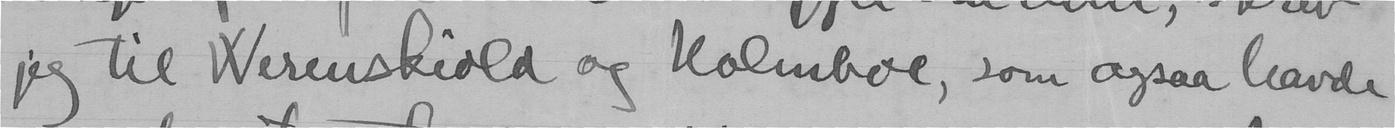jeg til Werenskiold og Holmboe, som ogsaa havde
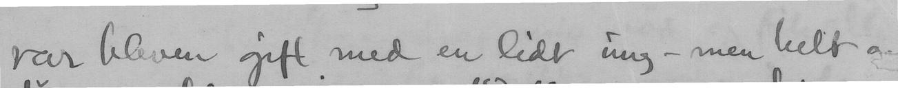var bleven gift med en lidt ung - men helt o
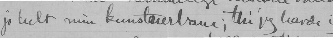jo helt min kunstnerbane; thi jeg havde i
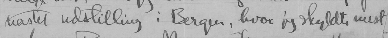hastet udstilling i Bergen, hvor jeg skyldte mest
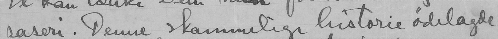raseri. Denne skammelige historie ødelagde
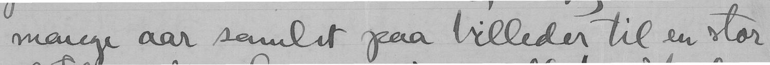mange aar samlet paa billeder til en stor
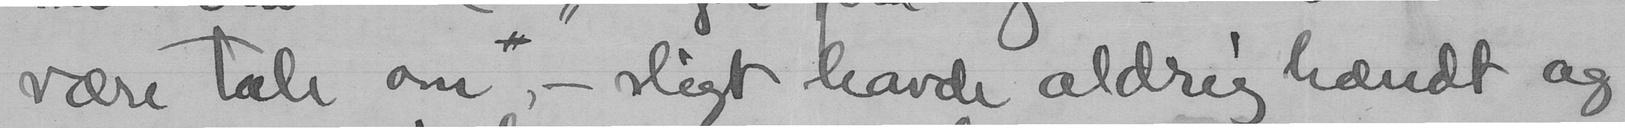være tale om", - sligt havde aldrig hændt og
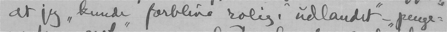at jeg "kunde forblive rolig i udlandet" - "penge-
